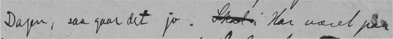Dagen, saa gaar det jo.  Har været paa
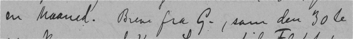en Maaned. Breve fra G., som den 30 te
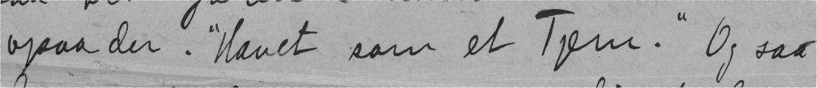ogsaa der. "Havet som et Tjern." Og saa
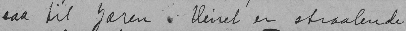saa til Jæren. Veiret er straalende
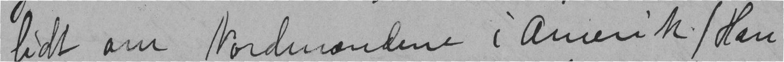lidt om Nordmændene i Amerik (Han
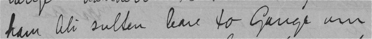ham bli sulten bare to Gange om
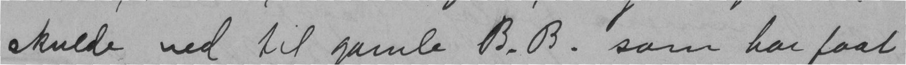skulde med til gamle B. B. som har faat
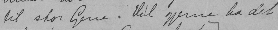til stor Gene. Vil gjerne ha det
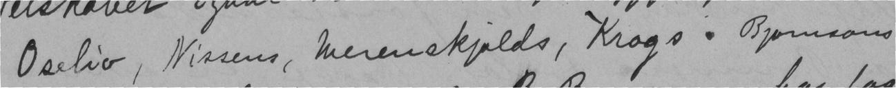Oselio, Nissens, Werenskjolds, Krogs. Bjørnsons
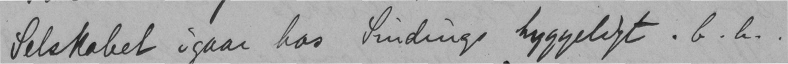Selskabet igaar hos Sindings hyggeligt. b.b.
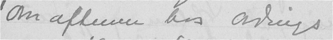Om aftenen hos Ordings
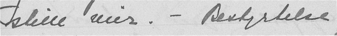stille veir. - Bestyrtelse
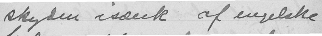skyden isænk af engelske
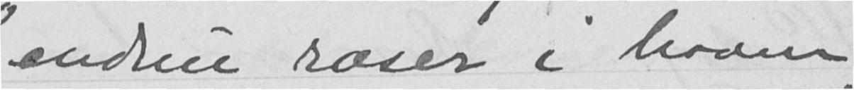endnu raser i haven
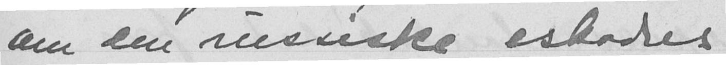om den russiske eskadres
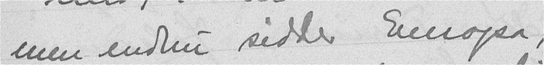men endnu sidder Europa,
